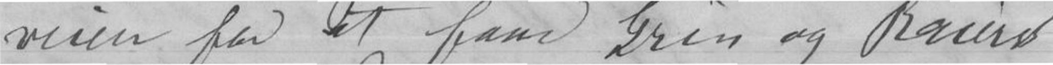veien for at faae Vin og Baier
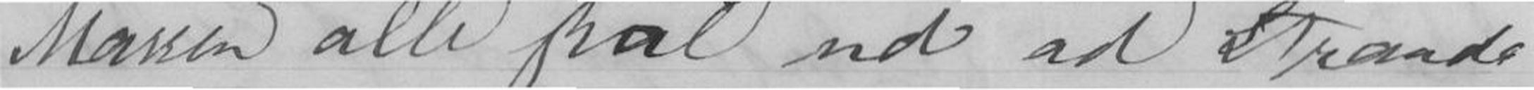Massen alle skal ud ad Strande-
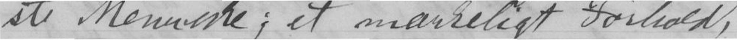ste Menneske; et mærkeligt Forhold,
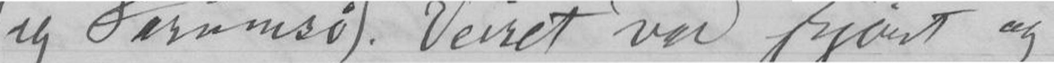og Ferumsø). Veiret var skjønt og
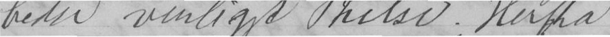beder venligst hilse. Herfra
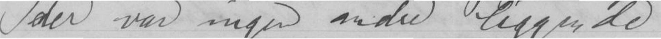der var ingen andre liggende
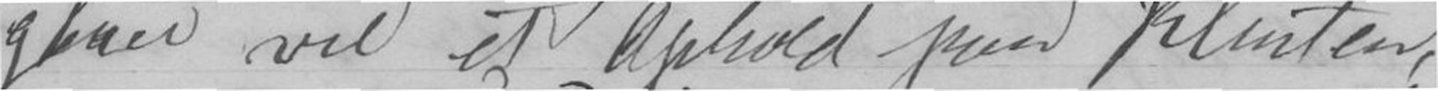gaaer vel et Ophold paa Klinten,
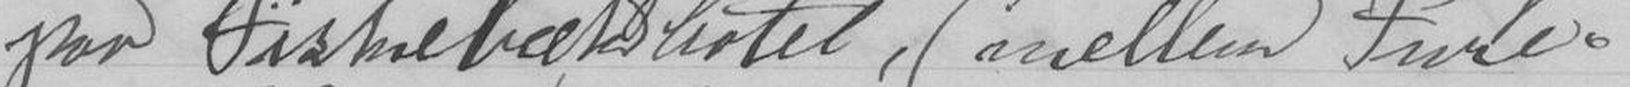paa Fiskelbækshotel, (mellem Fure
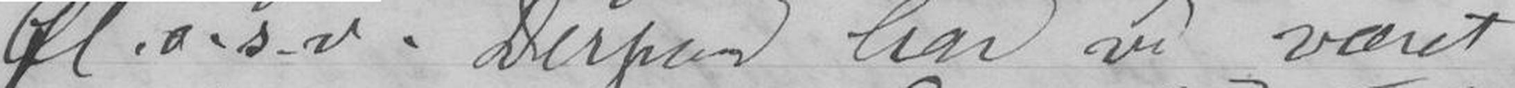Øl. o.s.v. Derpaa har vi været
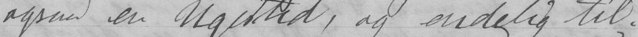ogsaa en Ugestid, og endelig til-
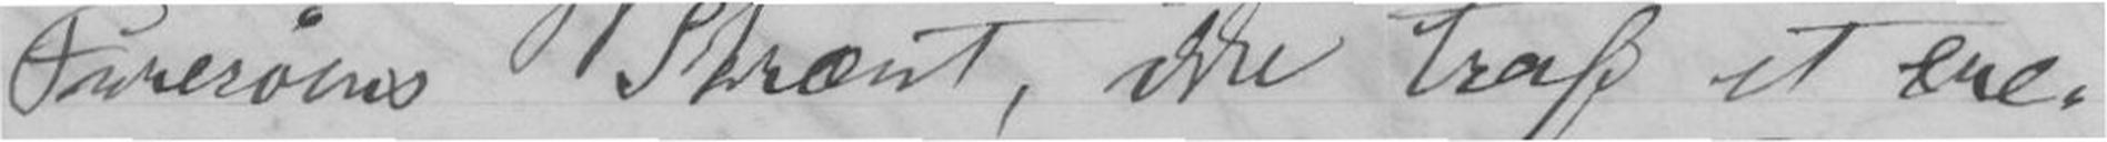Furesøens Skrænt, ikke traf et ene-
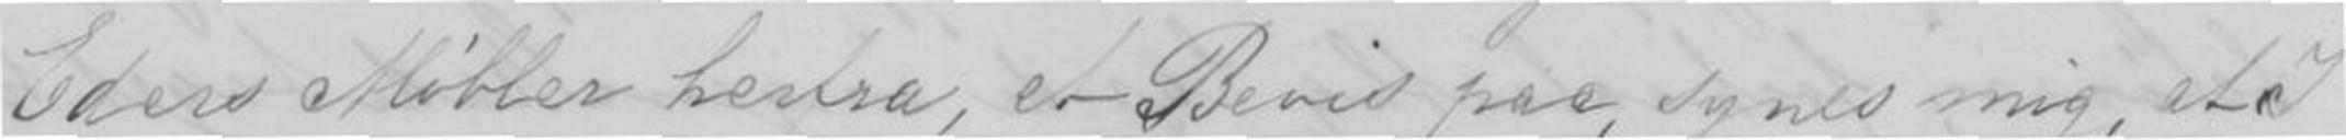Eders Møbler hefra, er Bevis paa, synes mig, at I
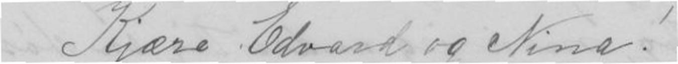Kjære Edvart og Nina!
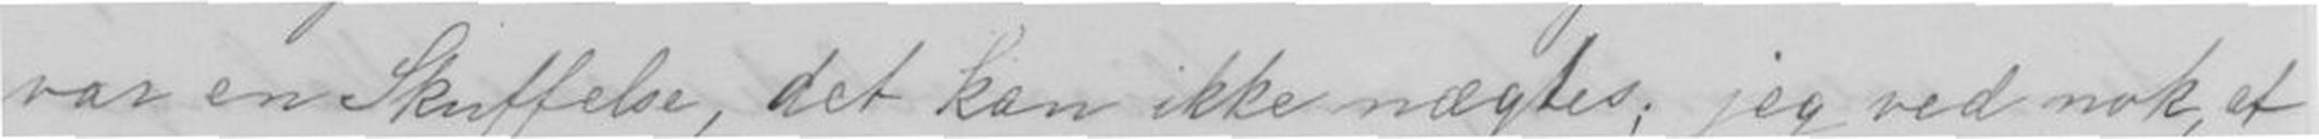var en Skuffelse, det kan ikke nægtes; jeg ved nok, at
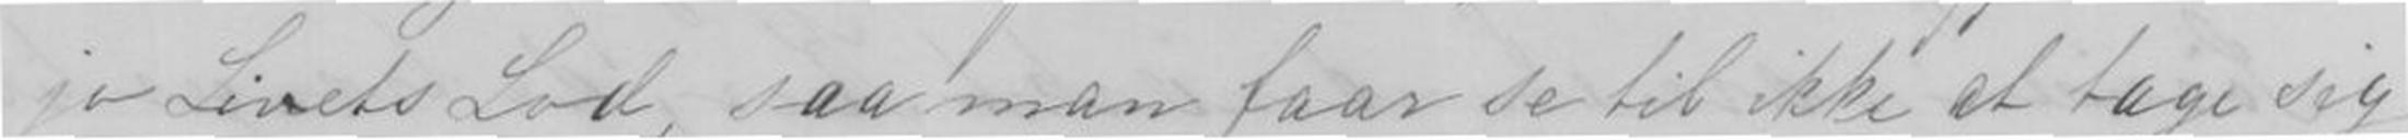jo Livets Lod, saa man faar se til ikke at tage sig
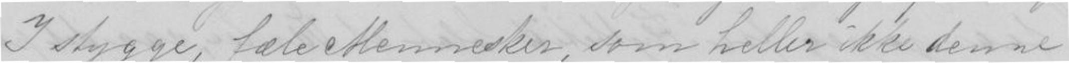I stygge, fæle Mennesker, som heller ikke denne
